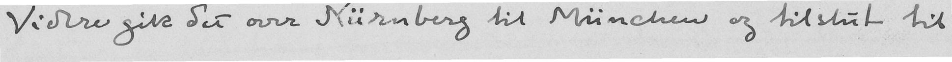Videre gik det over Nürnberg til München og tilslut til
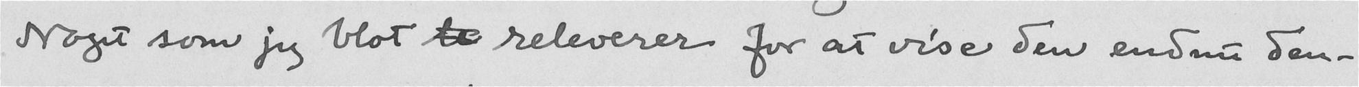Noget som jeg blot le releverer for at vise den endnu den-
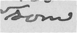som
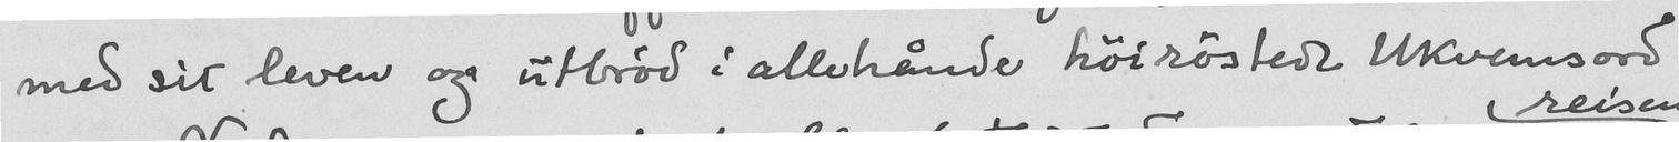med sit leven og utbrød i allehånde høirøstede Ukvemsord
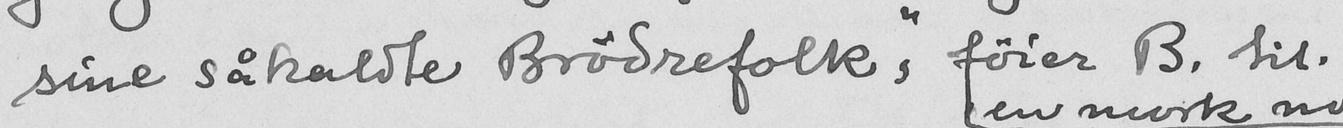sine såkaldte Brødrefolk," føier B. til.
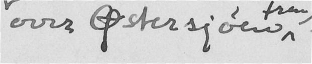over Østersjøen,
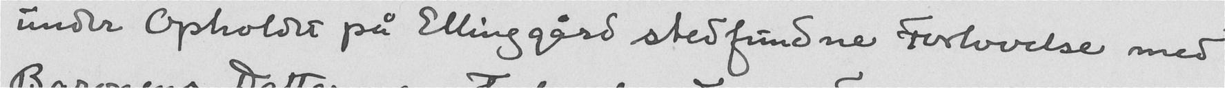under Opholdet på Ellinggård stedfundne Forlovelse med
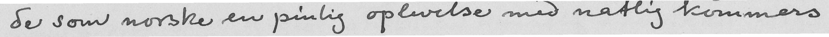de som norske en pinlig oplevelse med natlig kommers
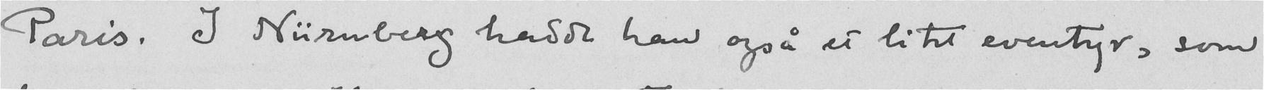Paris. I Nürnberg hadde han også et litet eventyr, som
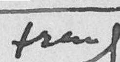fram
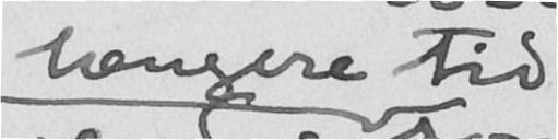længere tid
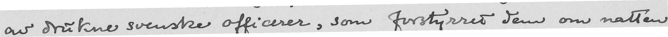av drukne svenske officerer, som forstyrret dem om natten
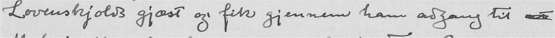Lovenskjolds gjæst og fik gjennem ham adgang til at
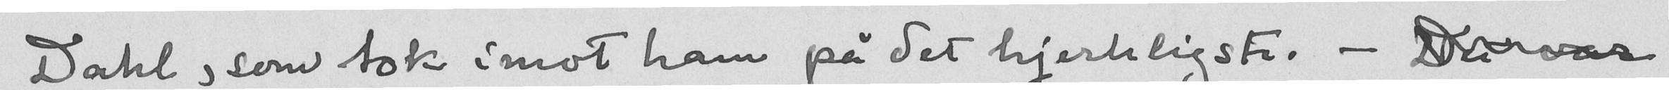Dahl, som tok imot ham på det hjerteligste. - Det var
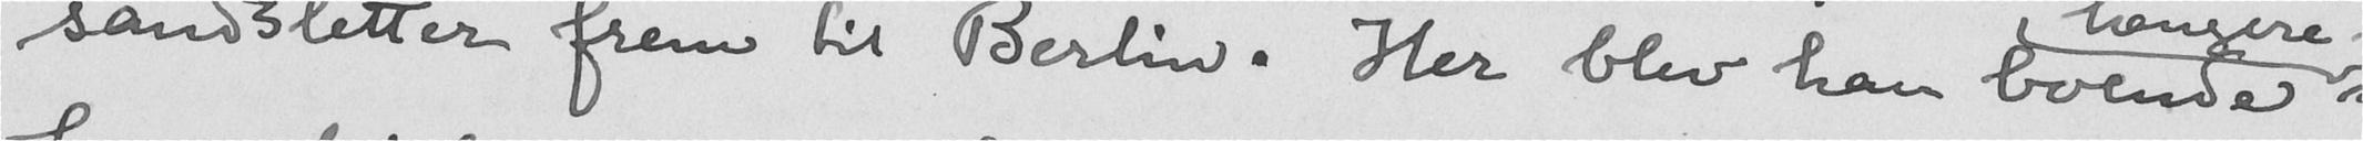sandsletter frem til Berlin. Her blev han boende
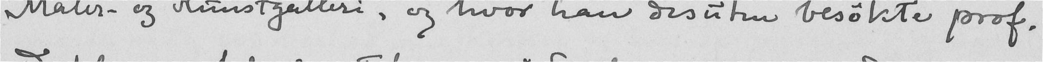Maler- og Kunstgalleri", og hvor han desuten besøkte prof.
